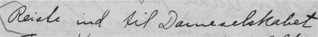Reiste ind til Dameselskabet
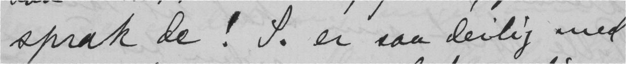sprak de! S. er saa derlig med
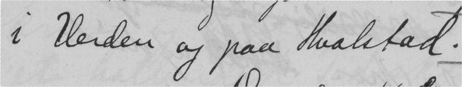i Verden og paa Hvalstad.
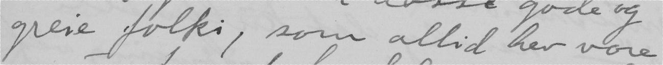greie folki, som altid hev vore
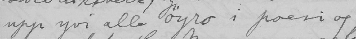upp yvi alle öyro i poesi og
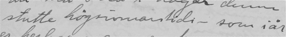stutte högsumarstidi – som iår
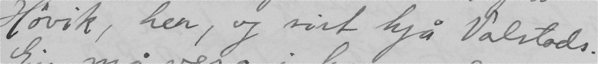Höøvik, her, og sist hjå Valstads.
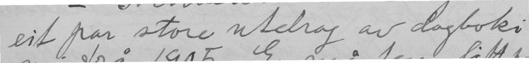eit par store utdrag av dagboki
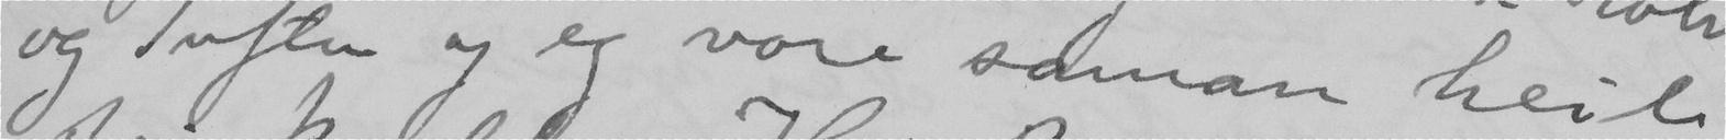og Tuften og eg vore saman heile
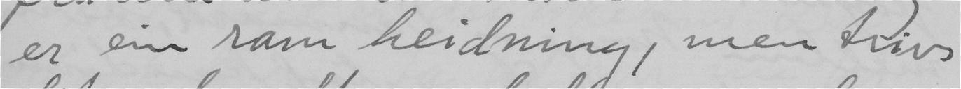er ein sann heidning, men trivs
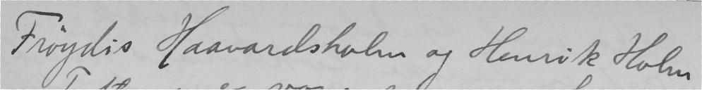Fröydis Haavardsholm og Henrik Holm
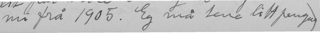nu frå 1905. Eg må tene litt pengar.
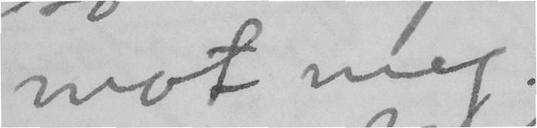mot meg.
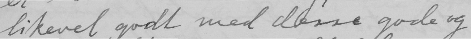likevel godt med desse gode og
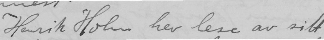Henrik Holm hev lese av sitt
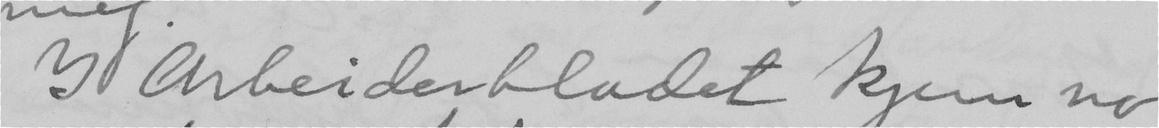I Arbeiderbladet kjem no
